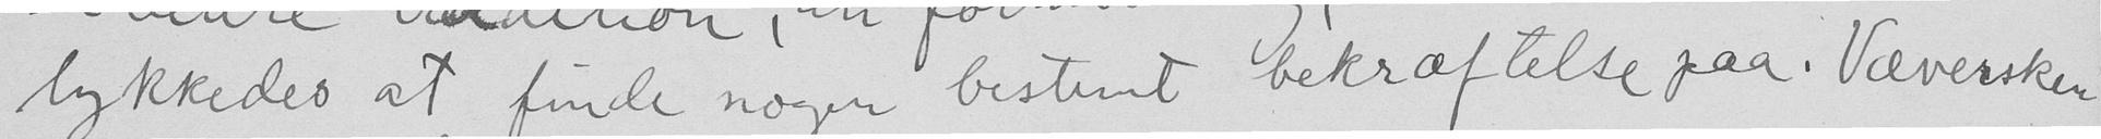lykkedes at finde nogen bestemt bekræftelse paa. Væversken
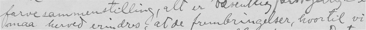maa herved erindres, at de frembringelser, hvortil vi
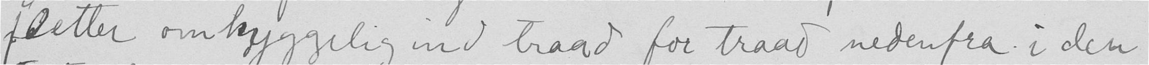fletter omhyggelig ind traad for traad nedenfra i den
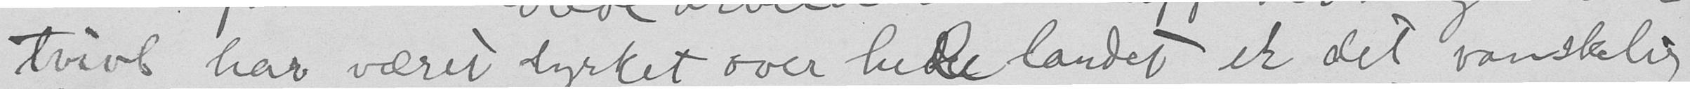tvivl har været dyrket over hele landet er det vanskelig
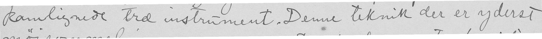kamlignede træ instrument. Denne teknik der er yderst
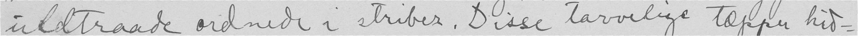uldtraade ordnede i striber. Disse tarvelige tæpper hid-
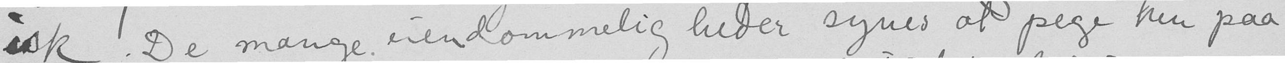isk. De mange eiendommeligheder synes av pege hen paa
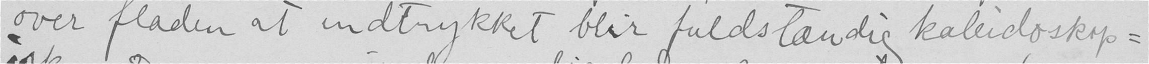over fladen at indtrykket blir fuldstændig kaleidoskop-
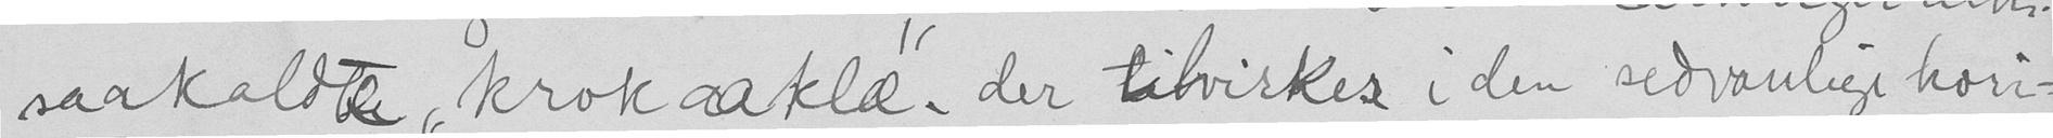saakaldte "krokaaklæ", der tilvirkes i den sedvanlige hori-
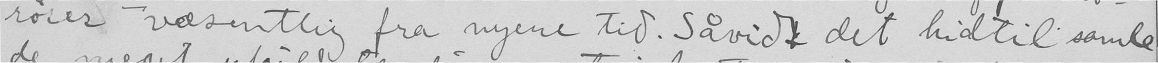røres væsentlig fra nyere tid. Såvidt det hidtil samle
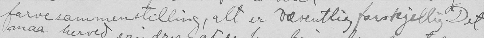farvesammenstilling, alt er væsentlig forskjellig. Det
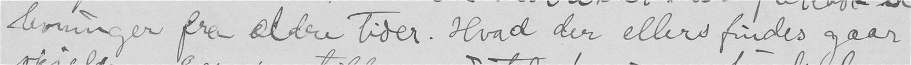levninger fra ældre tider. Hvad den ellers findes gaar
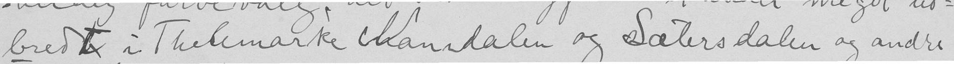bredt i Thelemarke Vanndalen og Sætersdalen og andre
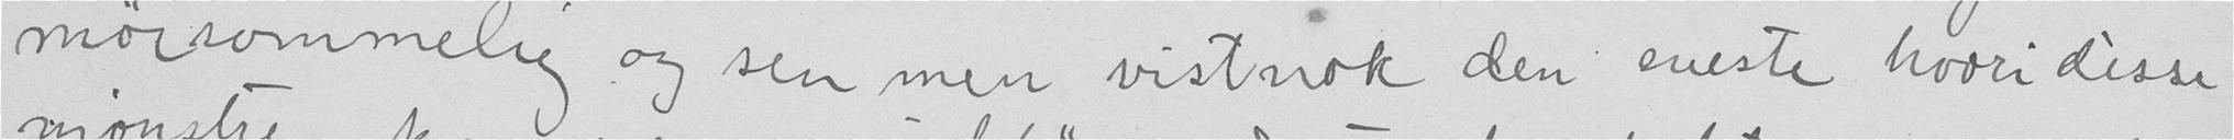møisommelig og sen men vistnok den eneste hvori disse
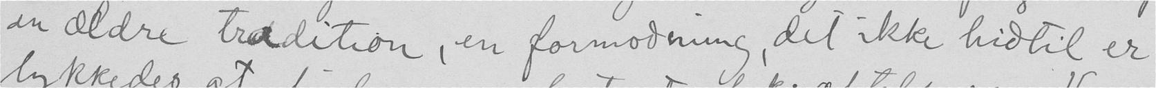en ældre tradition, en formodning, det ikke hidtil er
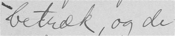betræk, og de
